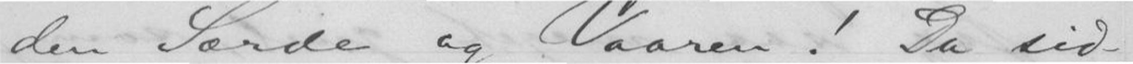den Særde og Vaaren! Da sid-
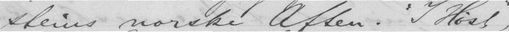steins norske Aften. "I Høst",
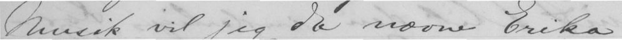Musik vil jeg da nævne Erika
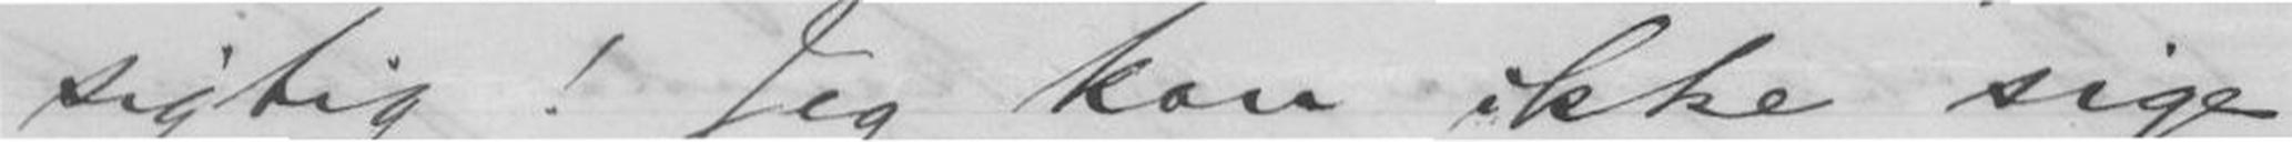sigtig! Jeg kan ikke sige
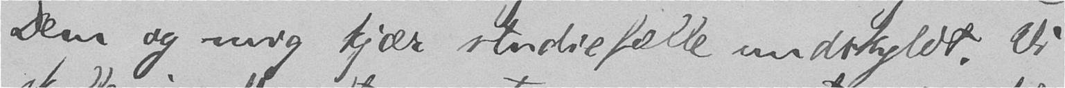Dem og mig kjær studiefælle undskyldt. Vi
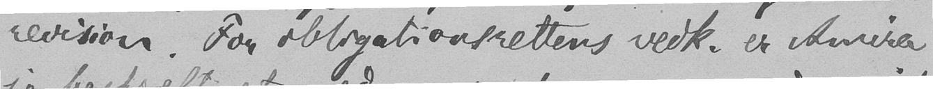revision. For obligationsrettens vedk. er Amira
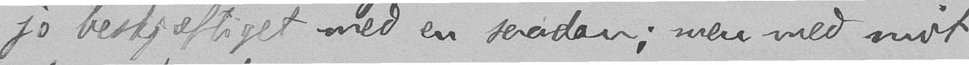jo beskjæftiget med en saadan; men med mit
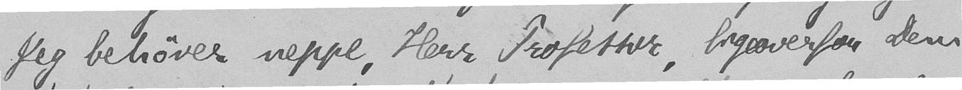Jeg behøver neppe, Herr Professor, ligeoverfor Dem
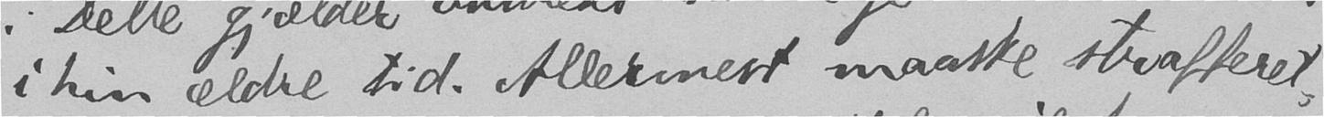i hin ældre tid. Allermest maaske strafferet-
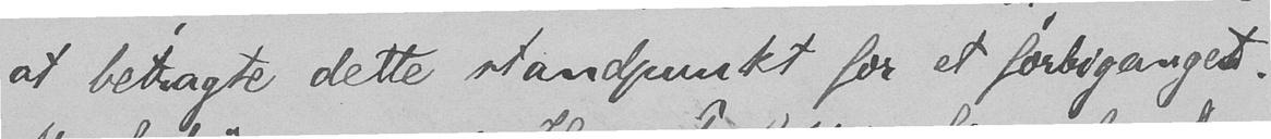at betragte dette standpunkt for et forbiganget.
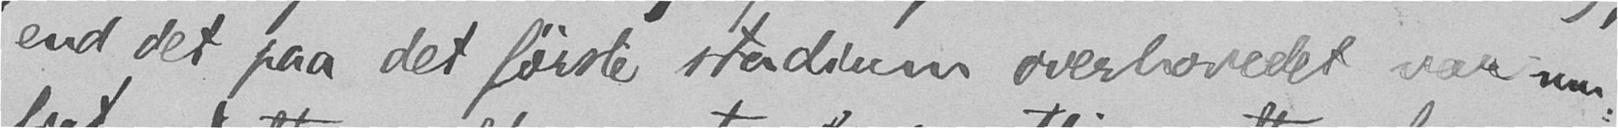end det paa det første stadium overhovedet var mu-
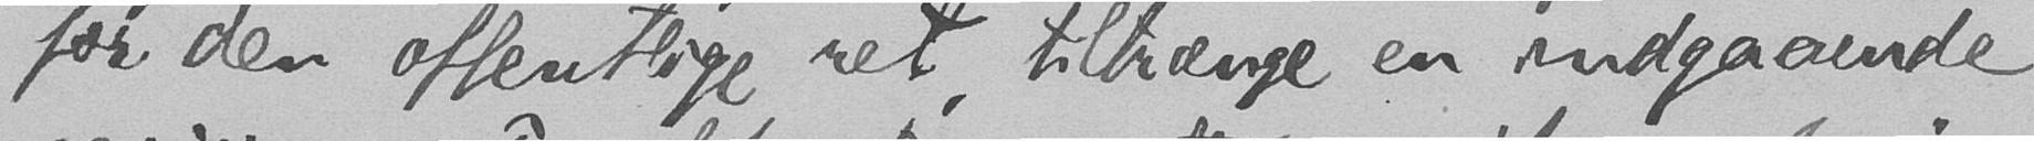for den offentlige ret, tiltrænge en indgaaende
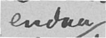endnu
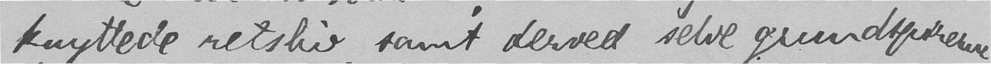knyttede retsliv, samt derved selve grundspirerne
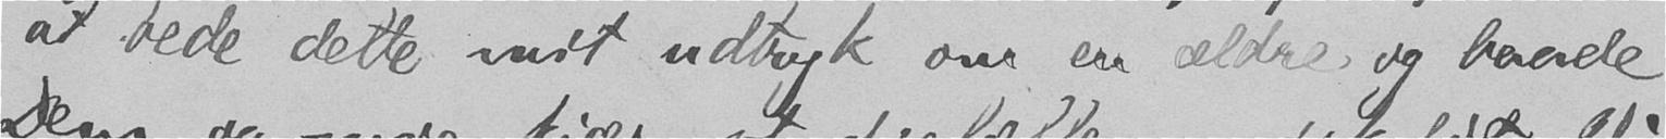at bede dette mit udtryk om en ældre og baade
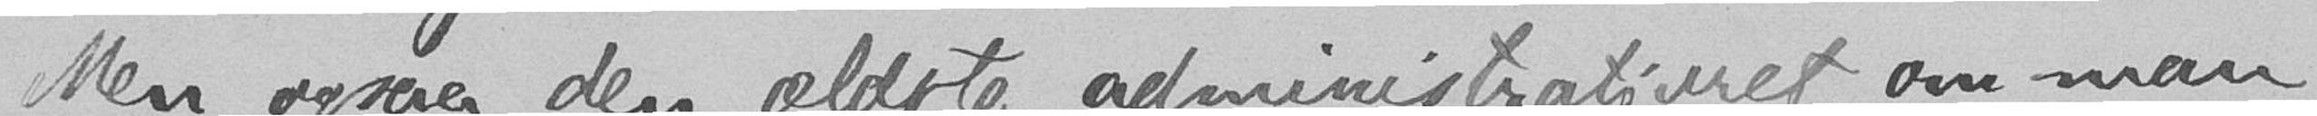Men ogsaa den ældste administrativret, om man
